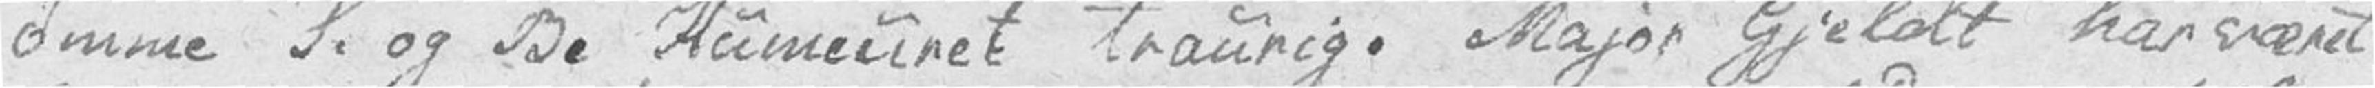ømme S. og B. Humeuret traurig. Major Gjeldt har været
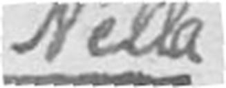Nella
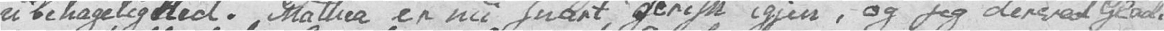ubehagelighed. Mathea er nu snart Frisk igjen, og jeg derved Glad.
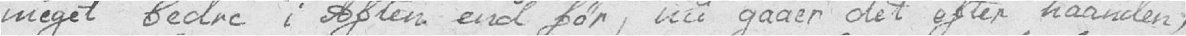meget bedre i Aften end før, nu gaaer det efter haanden,
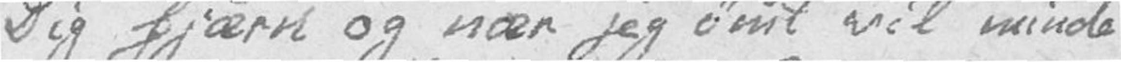Dig fjærn og nær jeg ømt vil minde
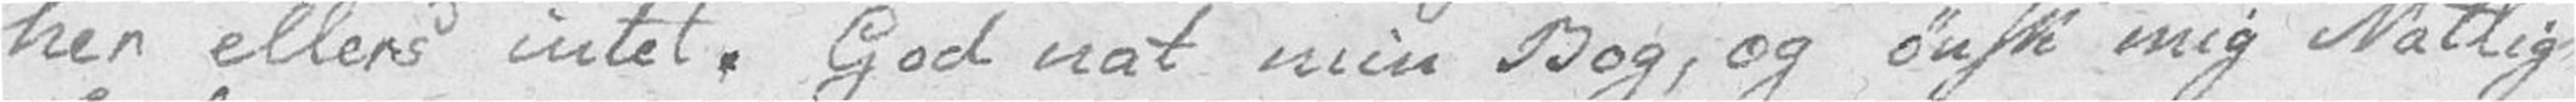her ellers intet. God nat min Bog, og ønsk mig Natlig
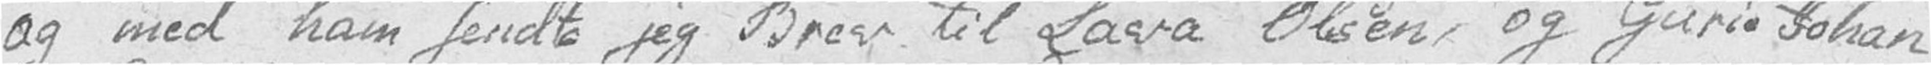og med ham sendte jeg Brev til Lava Olsen, og Guri Johan
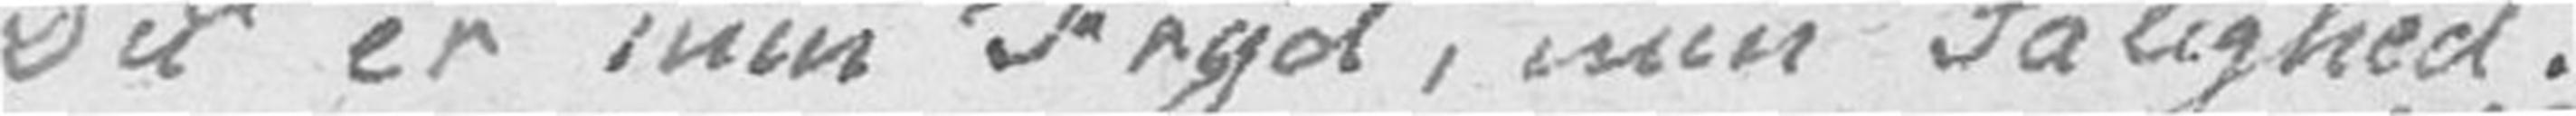Du er min Fryd, min Salighed.
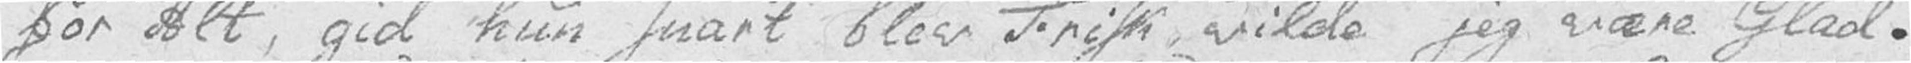for Alt, gid hun snart blev Frisk vilde jeg være Glad.
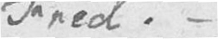Fred. –
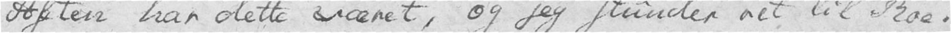Aften har dette været, og jeg stunder ret til Roe.
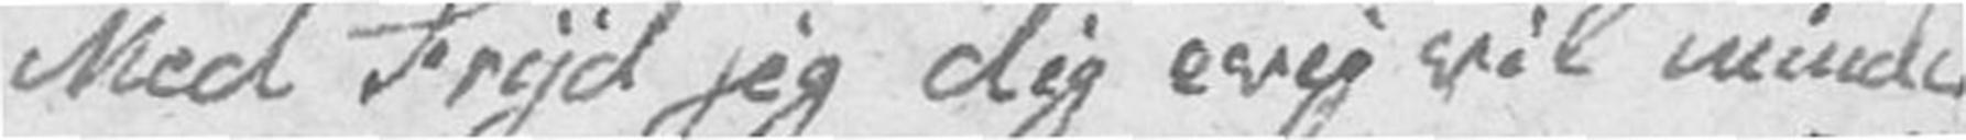Med Fryd jeg dig evig vil minde.
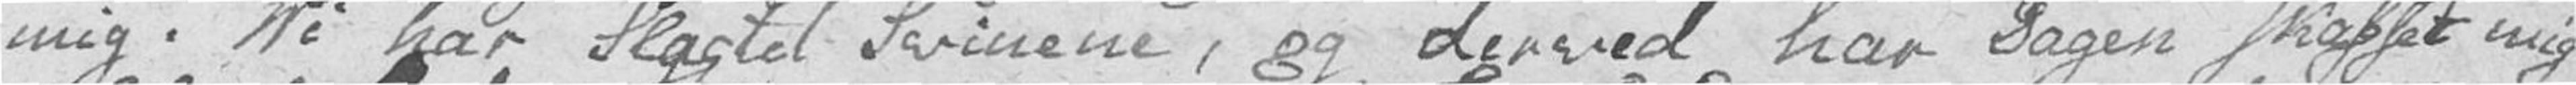mig. Vi har Slagtet Svinene, og derved har Dagen skaffet mig
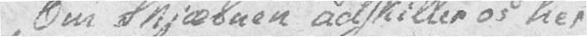Om Skjæbnen adskiller os her
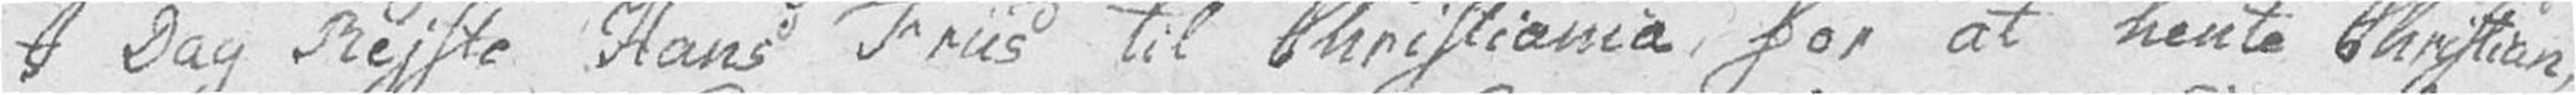I Dag Reiste Hans Friis til Christiania for at hente Christian,
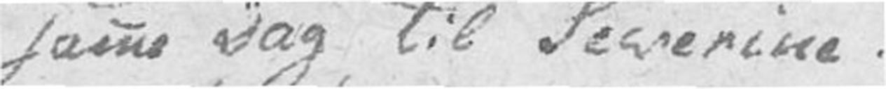samme Dag til Severine.
